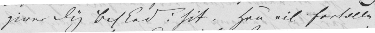giver Dig Besked i sit. Han vil fortælle
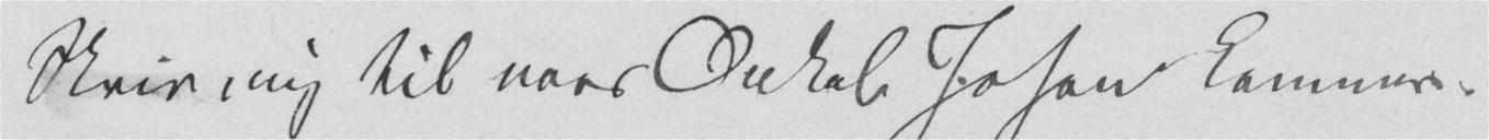Skriv mig til naar Onkel Johan kommer.
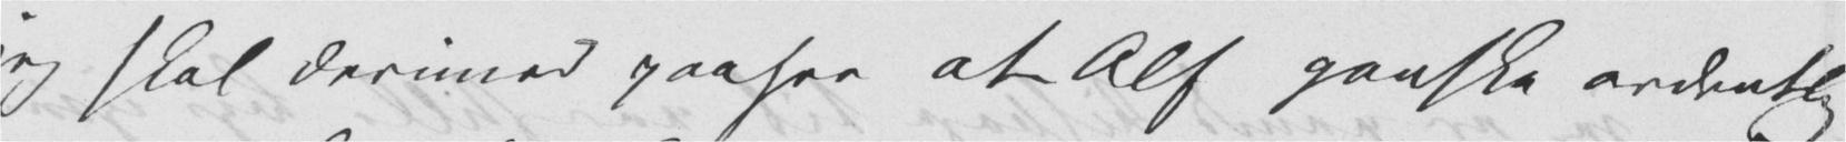jeg skal derimod paasee at Alf ganske ordentlig
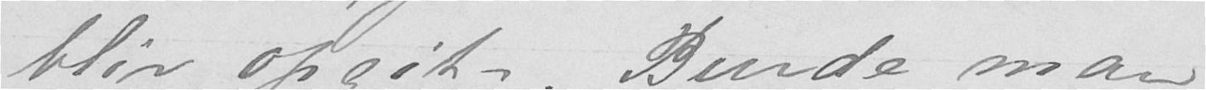blir opgit -. Burde man
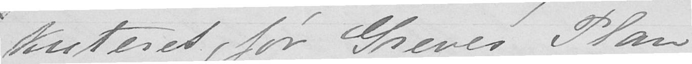kuteres, før Greves Plan
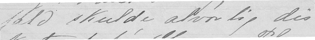fald skulde alvorlig dis-
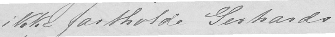ikke fastholde Gerhards
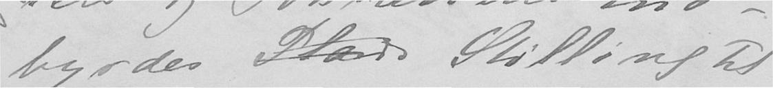byrdes Plads Stilling til
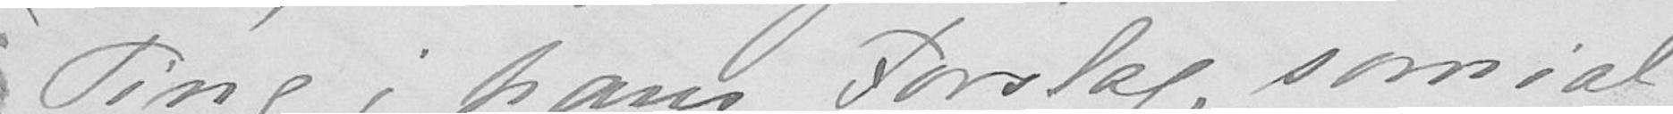Ting i hans Forslag, som ial-
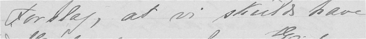Forslag, at vi skulde have
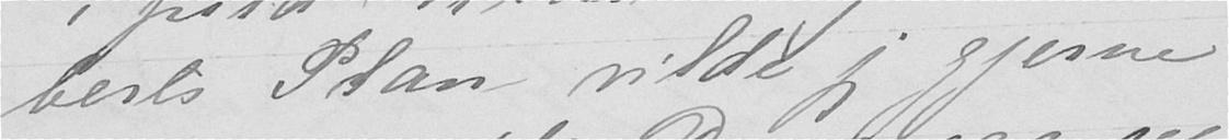berts Plan vilde jeg gjerne
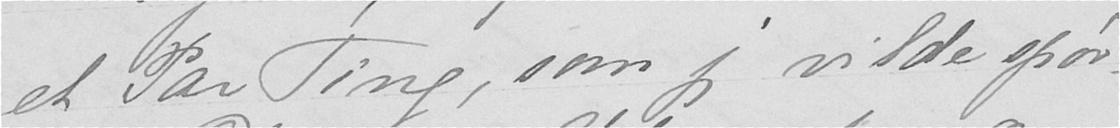et Par Ting, som jeg vilde spør-
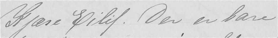Kjære Eilif. Der er bare
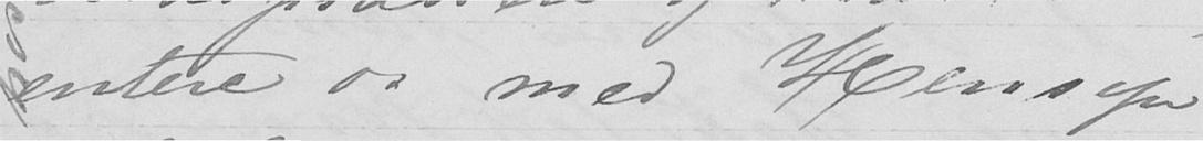entere os med Hensyn
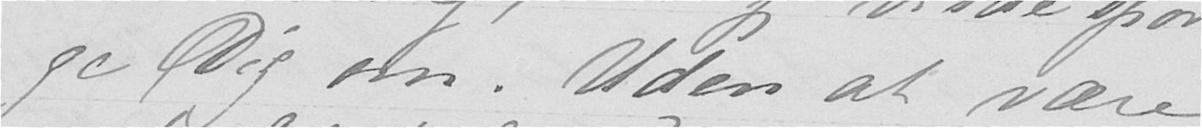ge Dig om. Uden at være
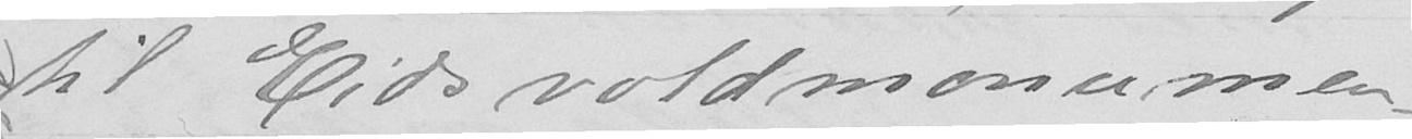til Eidsvoldmonumen-
